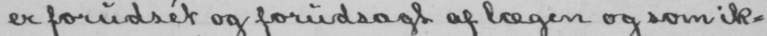er forudsét og forudsagt af lægen og som ik-
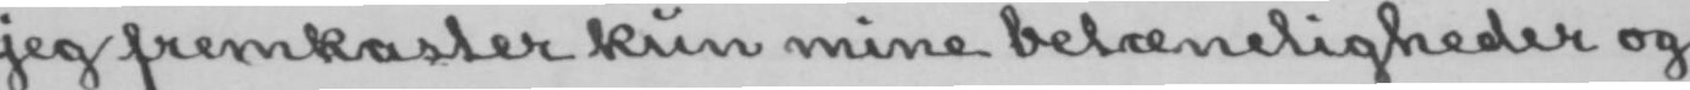jeg fremkaster kun mine betæneligheder og
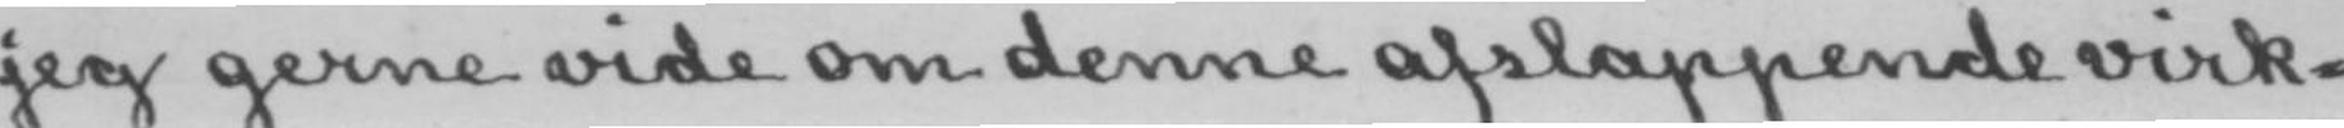jeg gerne vide om denne afslappende virk-
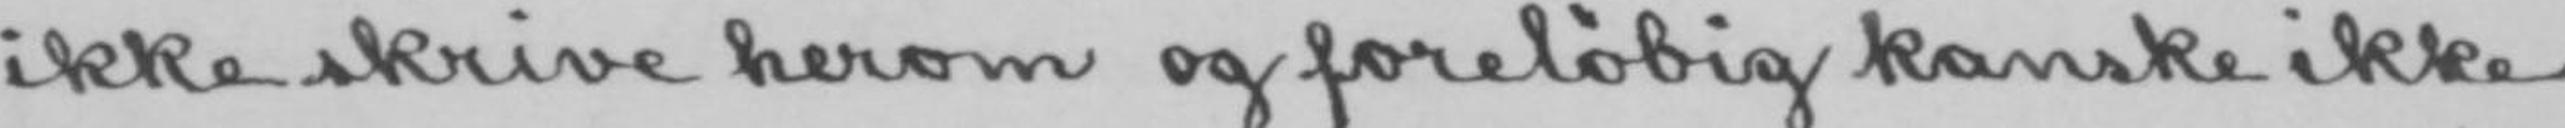ikke skrive herom og foreløbig kanske ikke
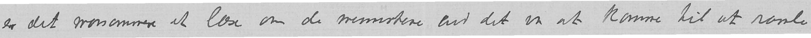er det morsommere at læse om de menneskene end det var at komme til at ramle
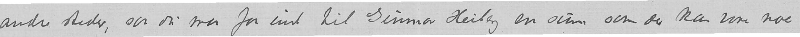andre steder, saa du maa faa in til Gunnar Heiberg en sum som der kan være noe
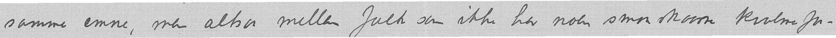samme emne, men altsaa mellem folk som ikke har noen smaaskaarne kvalme-
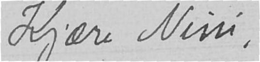Kjære Nini,
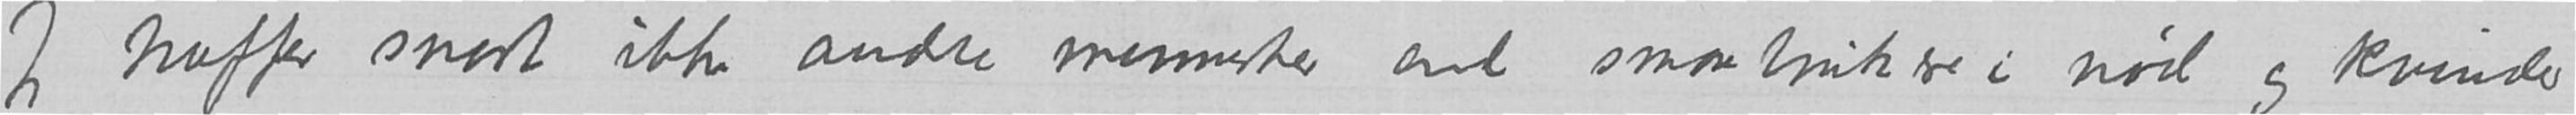Jeg træffer snart ikke andre mennesker end smaabrukere i nød og kvinder
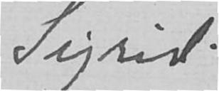Sigrid
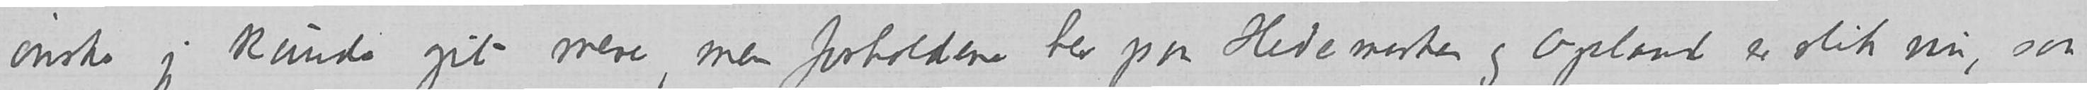ønske jeg kunde git mere, men forholdene her paa Hedemarken og Opland er slik nu, saa
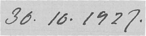30.10.1927
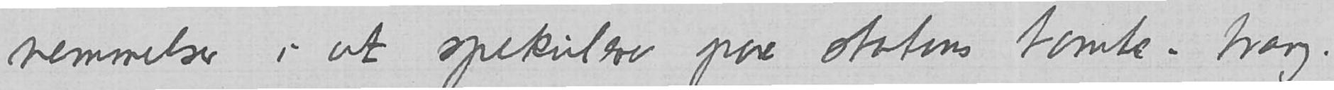fornemmelser i at spekulere paa statens tomte- tomte-trang.
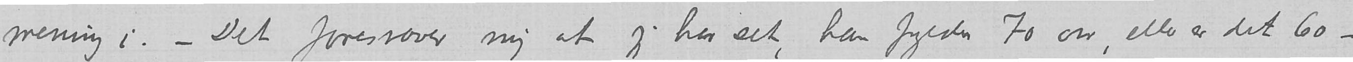mening i. – Det foresvever mig at jeg har set, han fylder 70 aar, eller er det 60
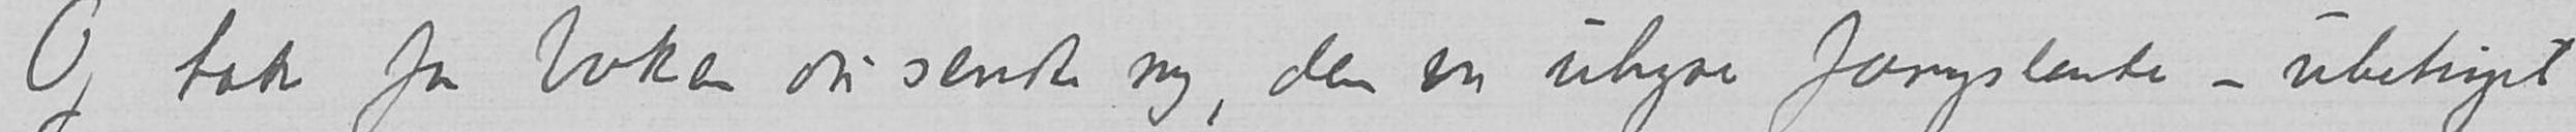Og tak for boken du sendte mig, den er uhyre fængslende – ubetinget
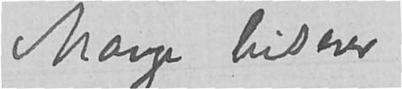Mange hilsener
